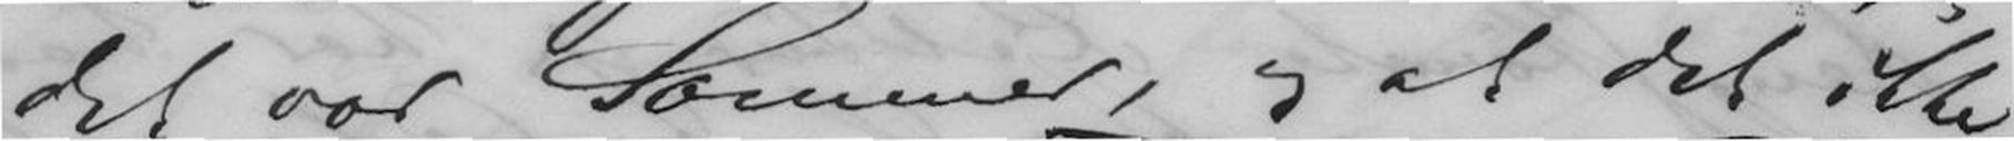det var Sommer, og at det ikke
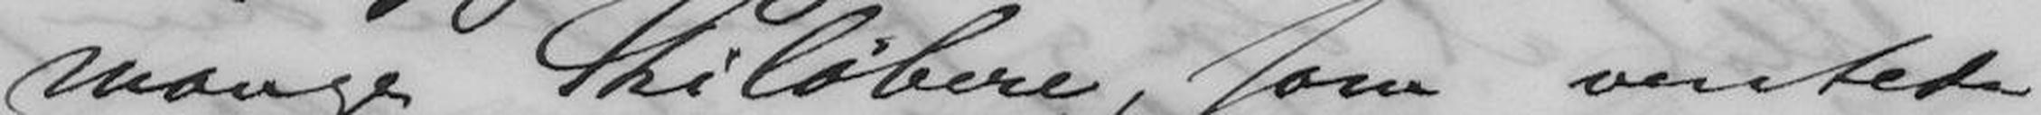mange Skiløbere, som ventede
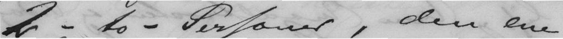2 - to - Personer, den ene
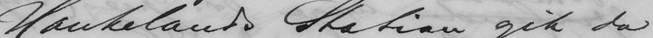Haukeland Station gik da
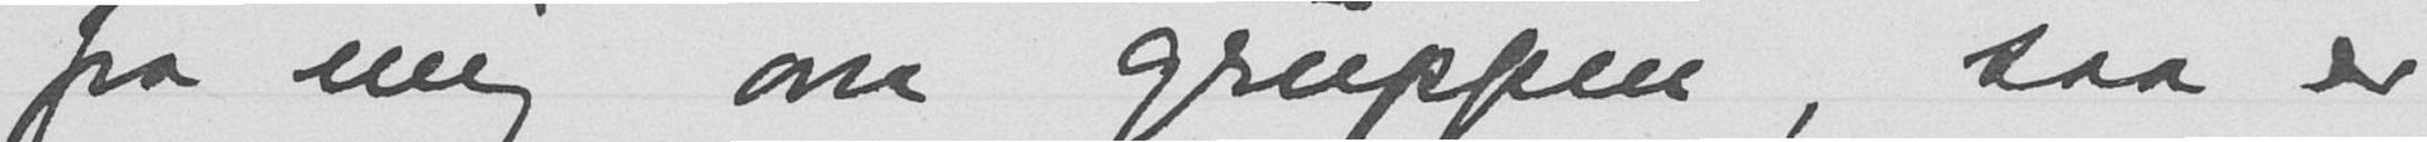fra mig om gruppen, saa er
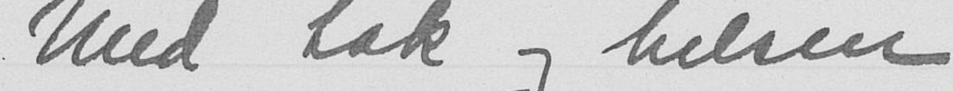Med tak og hilsen
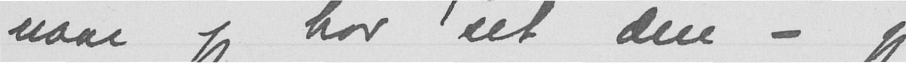naar jeg har set den - jeg
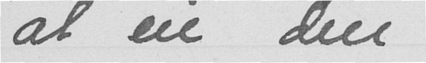at eie den.
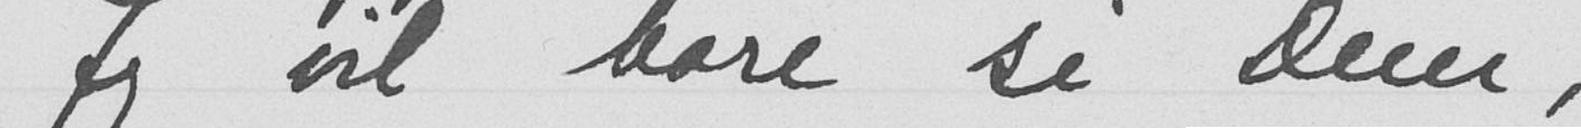Jeg vil bare si Dem,
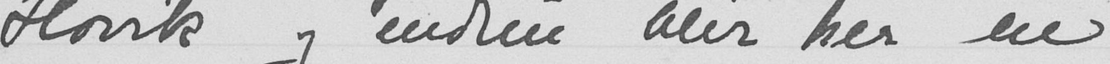Høvik og endnu blir her en
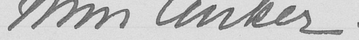Nini Anker.
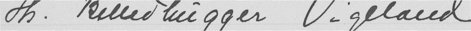Hr. Billedhugger Vigeland
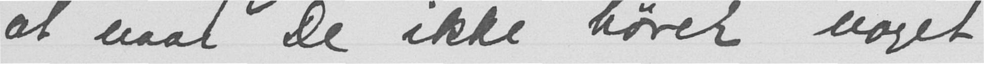at naar De ikke hører noget
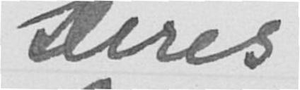Deres
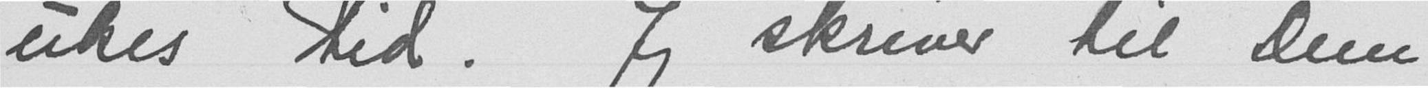ukes tid. Jeg skriver til Dem
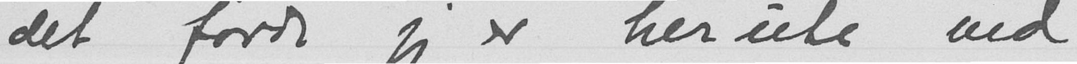det fordi jeg er her ute ved
